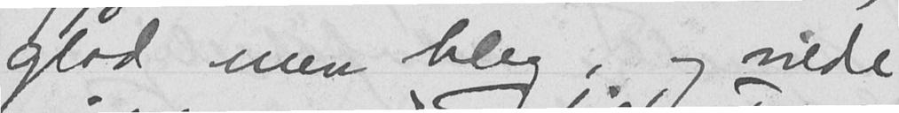glad men bleg, og vilde
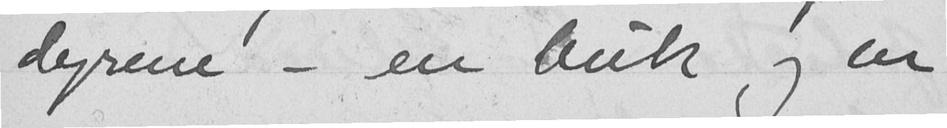dyrene - en buk og en
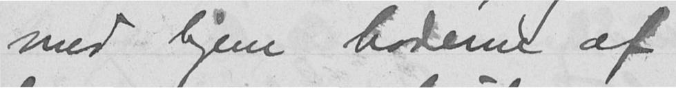med hjem hoderne af
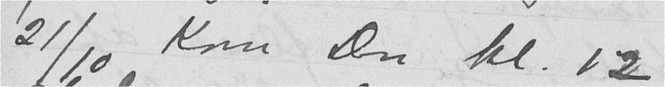21/10 Kom Don kl. 12,
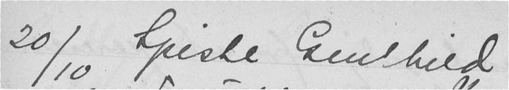20/10 Spiste Gunhild
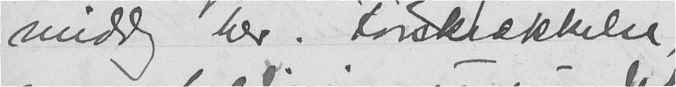middag her. Forskrækkelse,
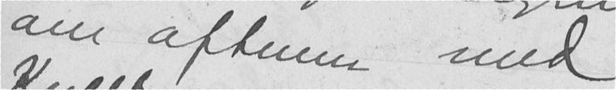om aftenen med
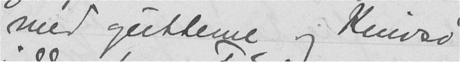med gutterne og Knivsø
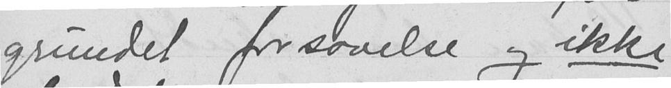grundet forsovelse og ikke
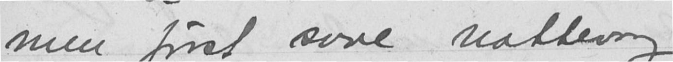men først sove nattevaag
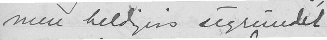men heldigvis ugrundet,
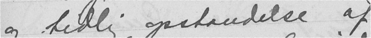og tidlig opstandelse af
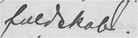fuldskab.
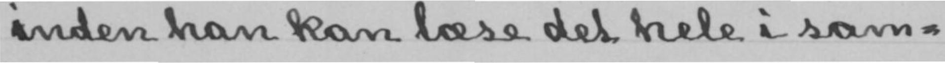inden han kan læse det hele i sam-
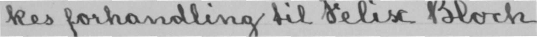kes forhandling til Felix Bloch
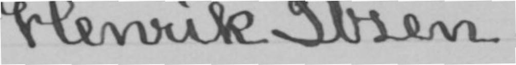Henrik Ibsen.
